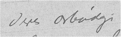Deres ærbødige
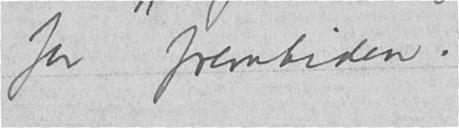for fremtiden.
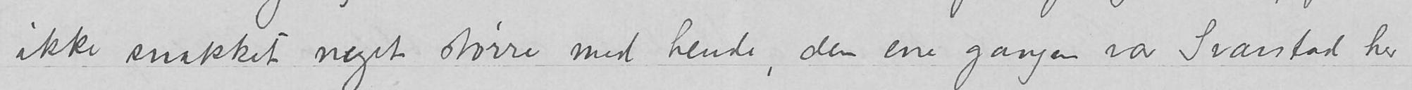ikke snakket noget større med hende, den ene gangen var Svarstad her
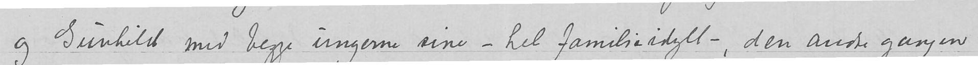og Gunhild med begge ungerne sine – hel familieidyll –, den andre gangen
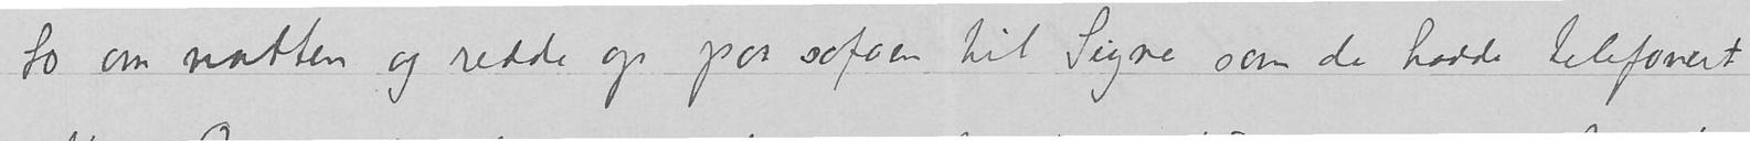to om natten og redde op paa sofaen til Signe som de hadde telefonert
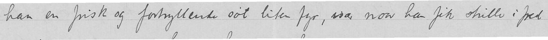han en frisk og fortryllende søt liten fyr, især naar han fik stille i fred
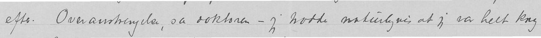efter. Overanstrengelse, sa doktoren – jeg trodde naturligvis at jeg var helt kng
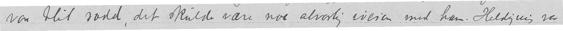var blit rædd, det skulde være noe alvorlig iveien med ham. Heldigvis var
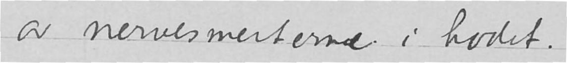av nervesmerterne i hodet.
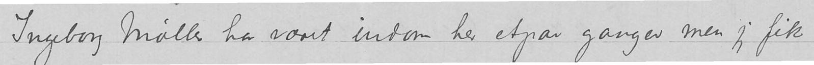Ingeborg Møller har været indom her etpar ganger men jeg fik
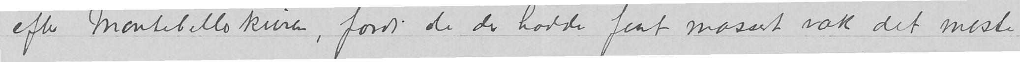efter Montebellokuren, fordi de der hadde faat massert væk det meste
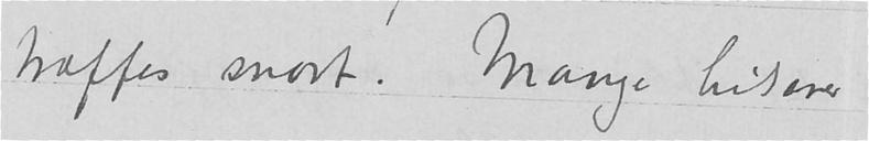træffes snart.  Mange hilsener
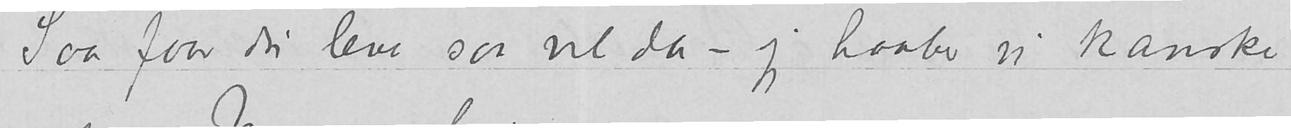Saa faar du leve saa vel da - jeg haaber vi kanske
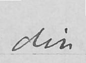din
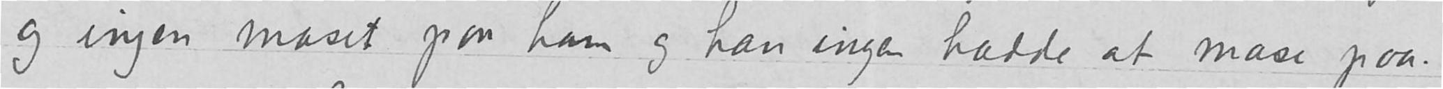og ingen maset paa ham og han ingen hadde at mase paa.
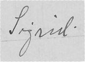Sigrid
